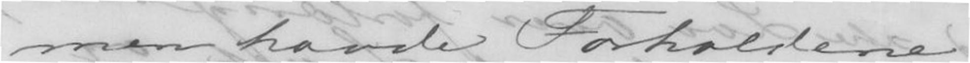men havde Forholdene
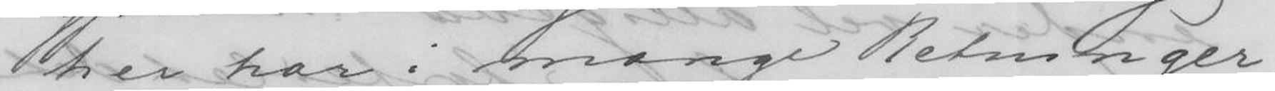her har i mange Retninger
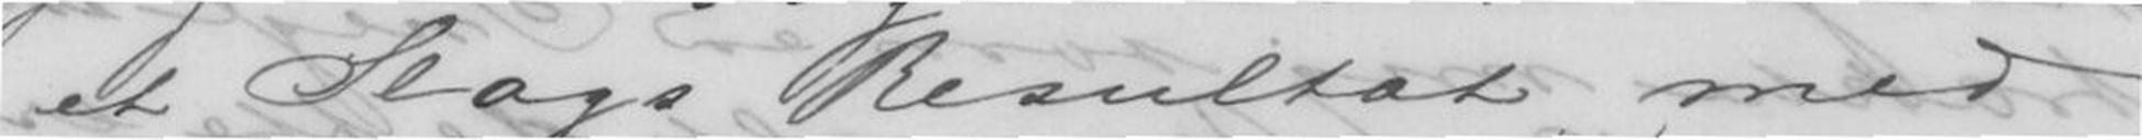et Slags Resultat med
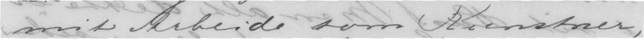mit Arbeide som Kunstner,
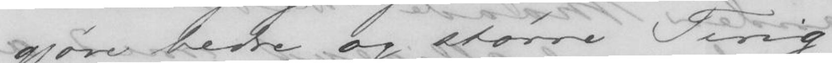gjøre bedre og større Ting,
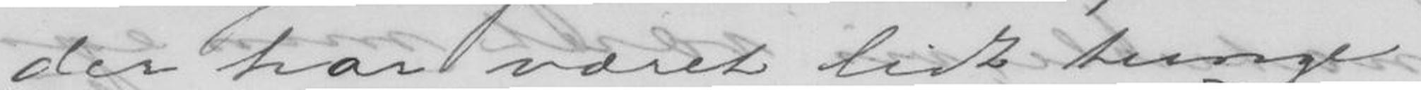der har været lidt tunge
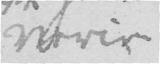Veeie
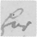for
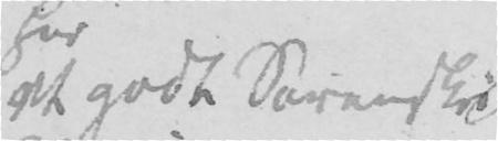et godt Sørenske
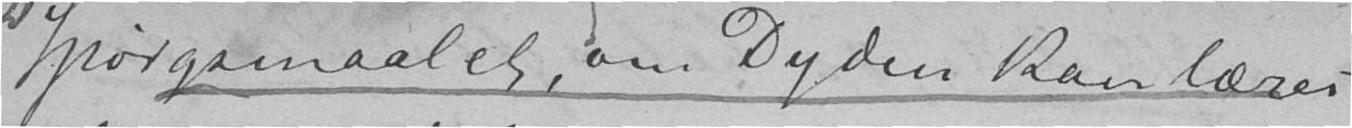Spørgsmaalet, om Dyden kan læres
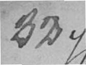32
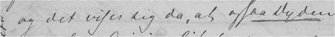og det viser sig da, at ogsaa Dyden
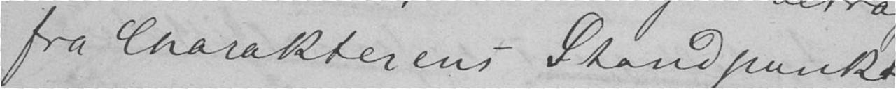fra Charakterens Standpunkt,
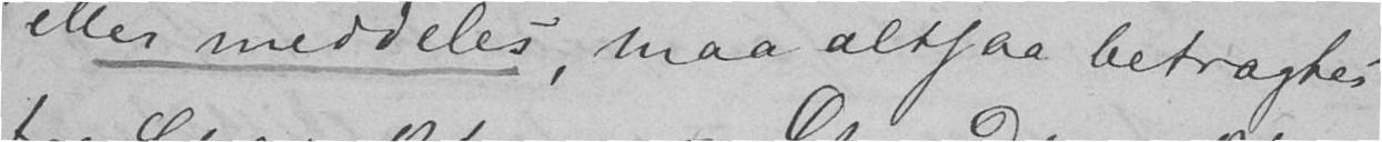eller meddeles, maa altsaa betragtes
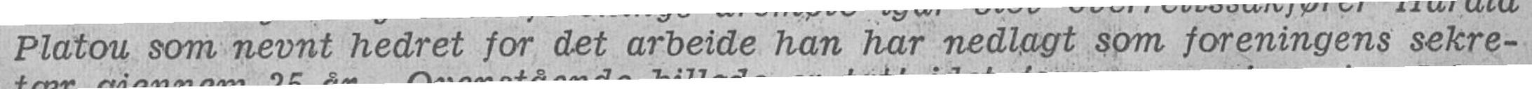Platou som nevnt hedret for det arbeide han har nedlagt som foreningens sekre-
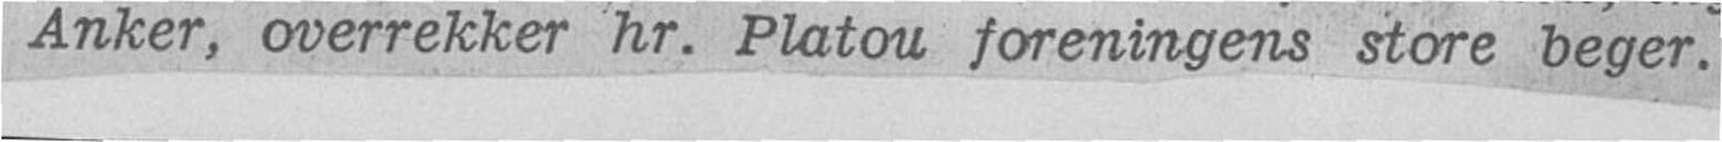Anker, overrekker hr. Platou foreningens store beger.
